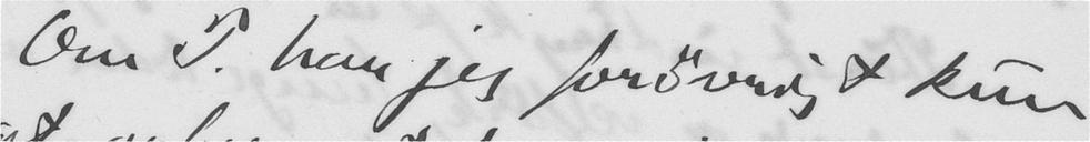Om P. har jeg forøvrigt kun
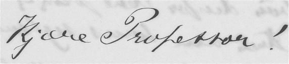Kjære Professor!
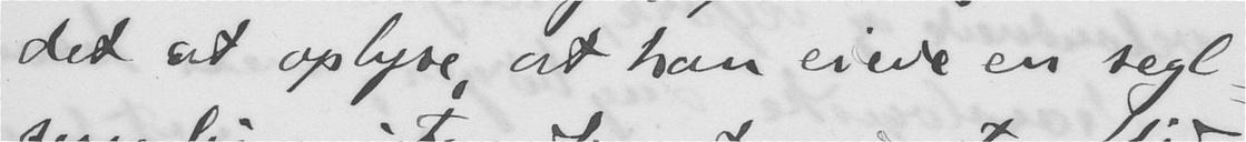det at oplyse, at han eiede en segl-
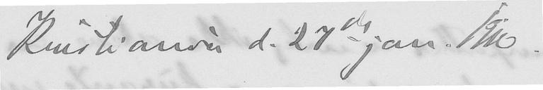Kristiania, d. 27de jan. 1910.
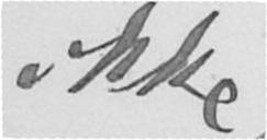ikke
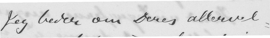Jeg beder om Deres allervel-
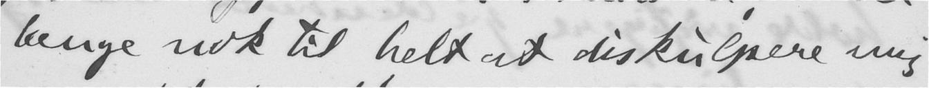længe nok til helt at diskulpere mig
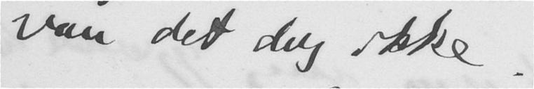var det dog ikke.
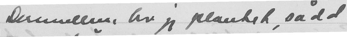Derimellom har jeg plantet, sådd
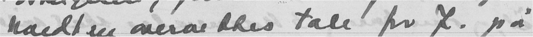holdt en overvetkes tale for J. på
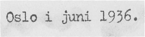Oslo i juni 1936
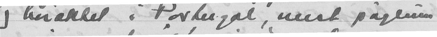og høiaktet i Portugal, mest pågrunn
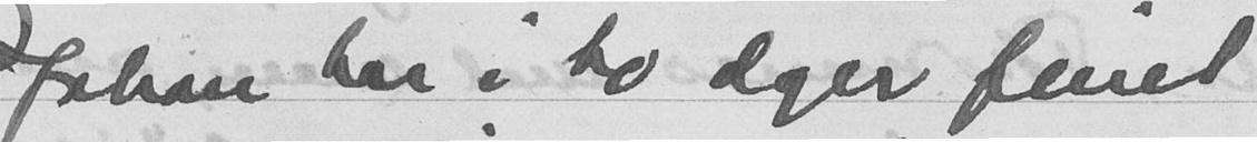Johan har i to dager feiret
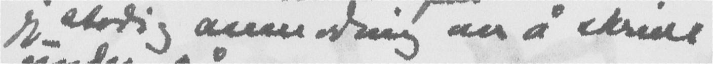jeg stadig anmoding om å skrive
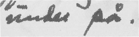under på.
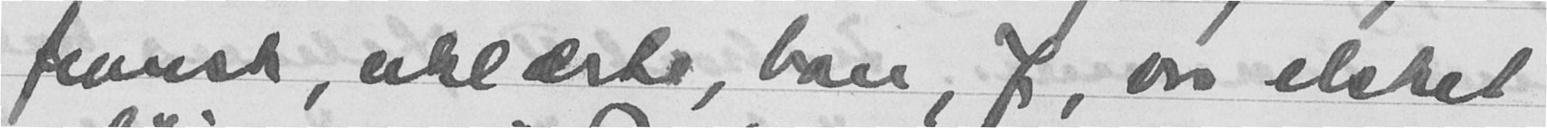fransk, erklærte, han, J, er elsket
